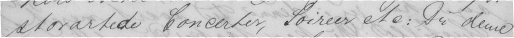storartede Concerter, Soireer etc: Du denne
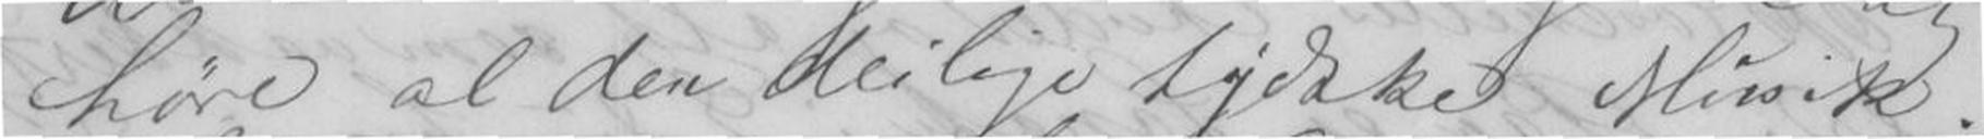høre al den deilige tydske Musik.
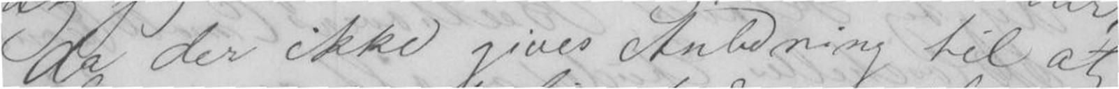da der ikke gives Anledning til at
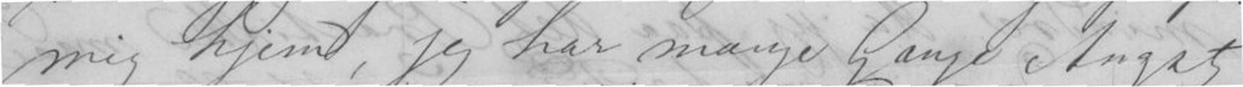mig hjem, jeg har mange Gange Angst
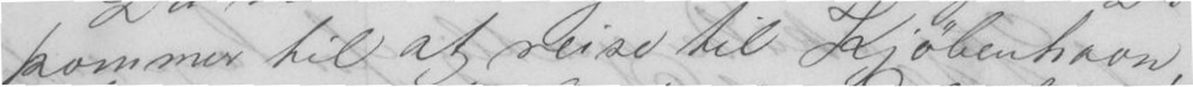kommer til at reise til Kjøbenhavn,
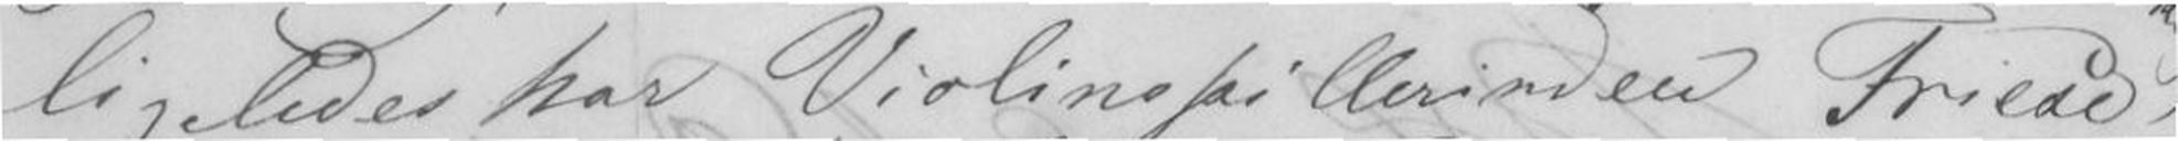ligeledes har Violinspillerinden Friede,
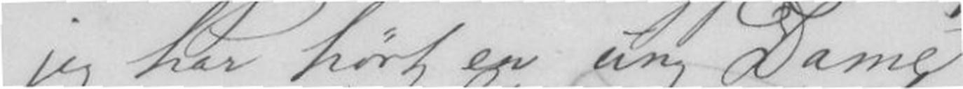jeg har hørt en ung Dame
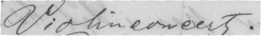Violinconcert.
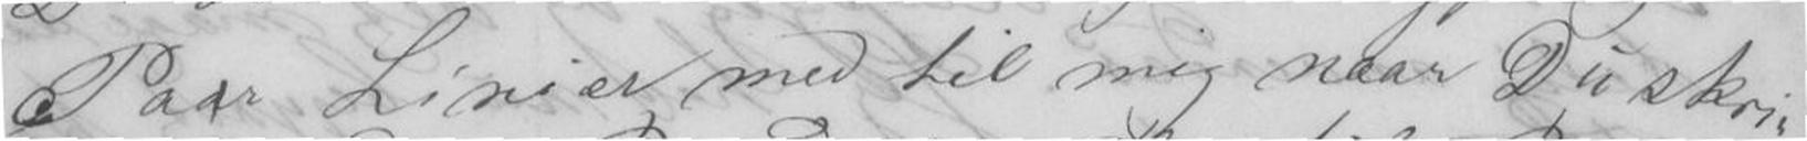Par Linier med til mig naar Du skri-
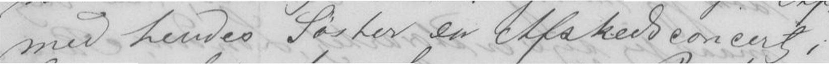med hendes Søster en Afskedsconcert i
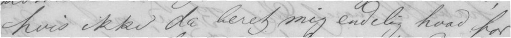hvis ikke da beret mig endelig hvad for
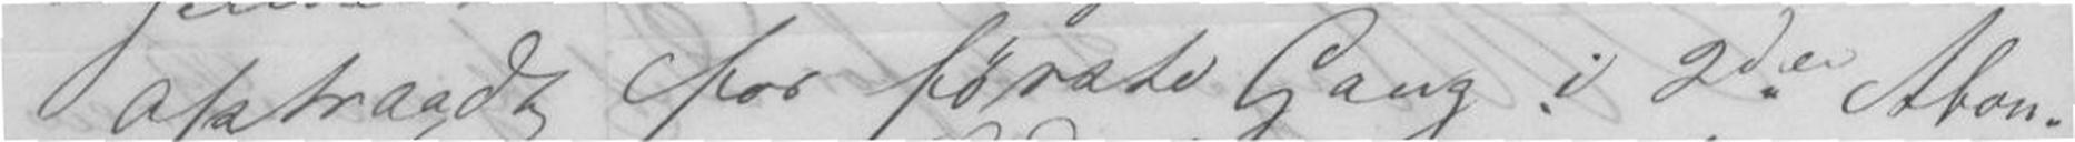optraadt for første Gang i 2den Abon-
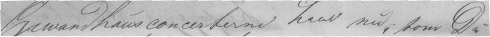Gewanthausconcerterne har nu, som Du
